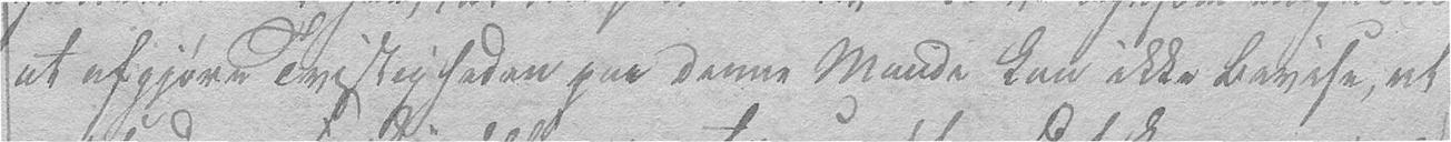at afgjøre Tvistigheden paa denne Maade kan ikke bevise, at
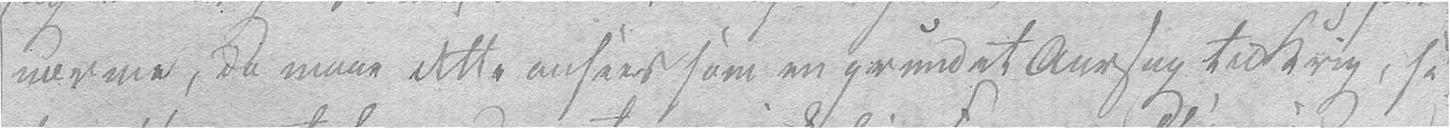nærme, da maa dette ansees som en grundet Aarsag til Krig, si-
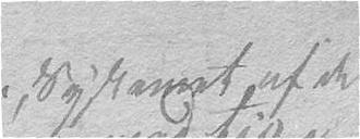Systemet af de
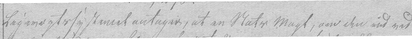Ligevægtssystemet antager, at en Stats Magt, om den end ved
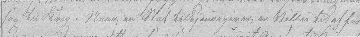sag til Krig. Naar en Stat tilkjendegiver en Villie til at for-
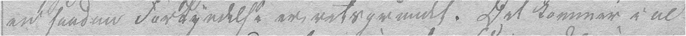en saadan Forkyndelse er retsgrundet. Det kommer i al
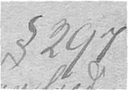§ 297.
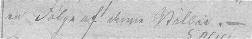en Følge af denne Villie. –
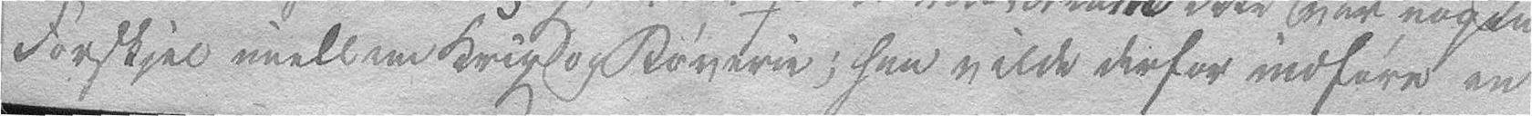Forskjel mellem Krig og Røverie; han vilde derfor indføre en
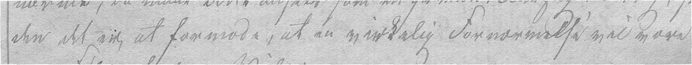den det er at formode, at en virkelig Fornærmelse vil være
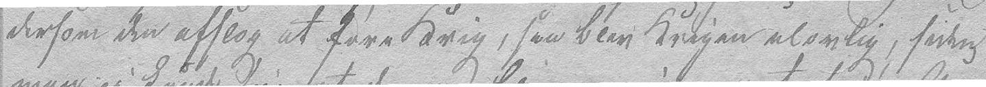dersom den afslog at føre Krig, saa blev Krigen ulovlig, siden
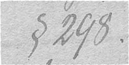§ 298.
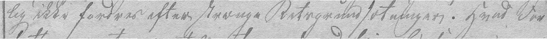lig ikke fordres efter strænge Retsgrundsætninger. Hvad For-
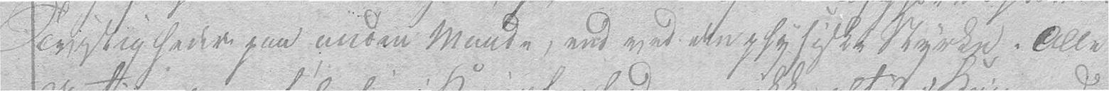Tvistigheder paa anden Maade, end ved den physiske Styrke. Alle
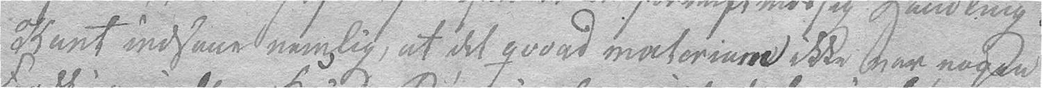Kant indsaae nemlig, at det qvoad materiam var nogen
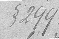§ 299.
